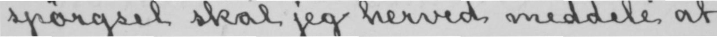spørgsel skal jeg herved meddele at
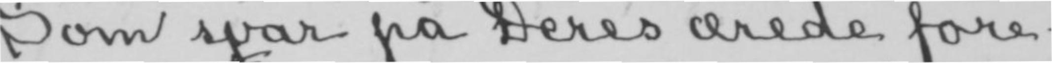Som svar på Deres ærede fore-
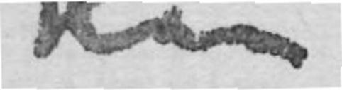kan
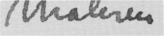Maleren
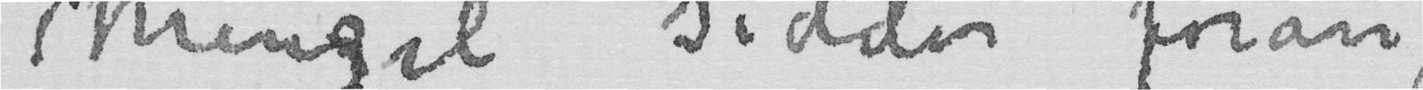Menzel sidder foran
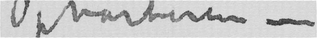Opvarteren –
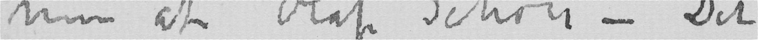men af Olaf Schou – Det
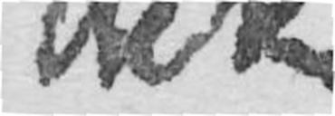ikke
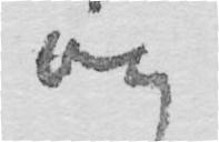og
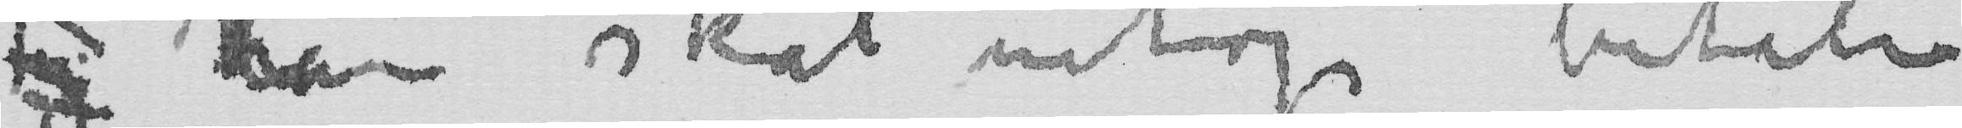Jeg han skal netop betale
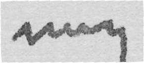mig
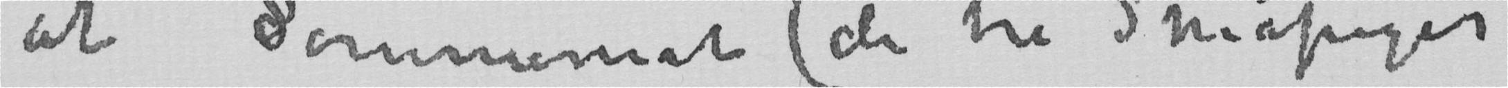at Sommernat (de tre Småpiger
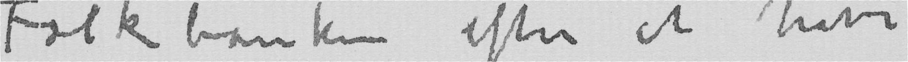Folkebanken efter at have
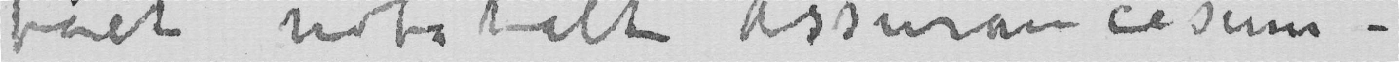fået udbetalt Assurancesum-
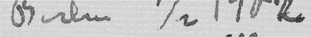Berlin 7/2 1902
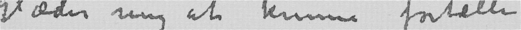glæder mig at kunne fortælle
